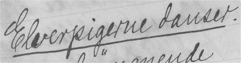Elverpigerne danser.
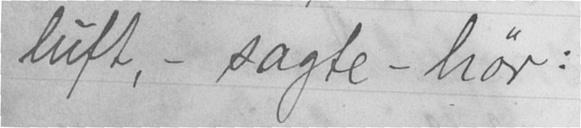luft, - sagte - hør:
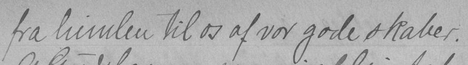fra himlen til os af vor gode skaber.
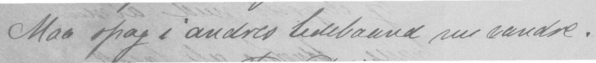Maa spag i andres belebanna nu vandre.
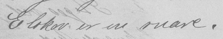Elskov er en snare.
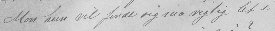Mon hun vil finde sig saa rigtig let i
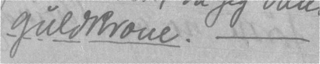guldkrone. -
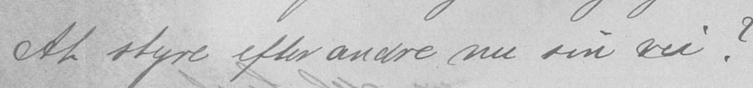At styre efter andre nu sin vei?
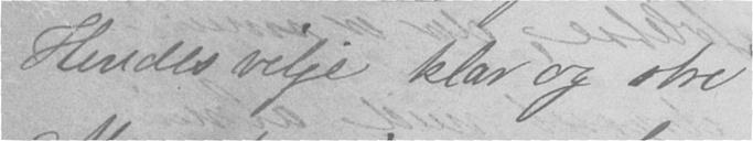Hendes vilje klar og stri
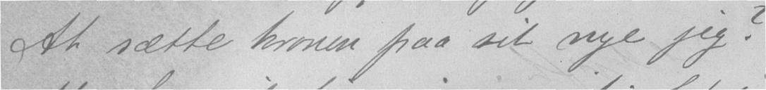At sætte kronen paa sit nye jeg?
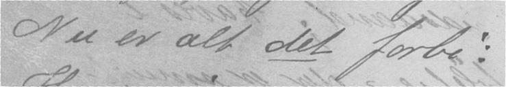Nu er alt det forbi:
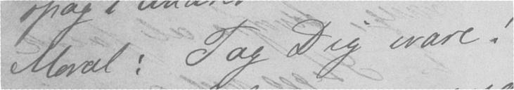Moral: Tag Dig ivare!
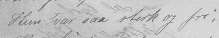Hun var saa sterk og fri;
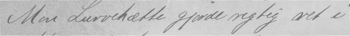Min Lurvehætte gjorde rigtig ret i
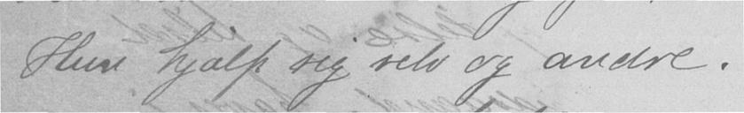Hun hjalp sig selv og andre.
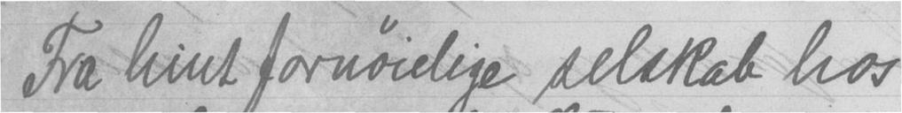Fra hint fornøielige selskab hos
